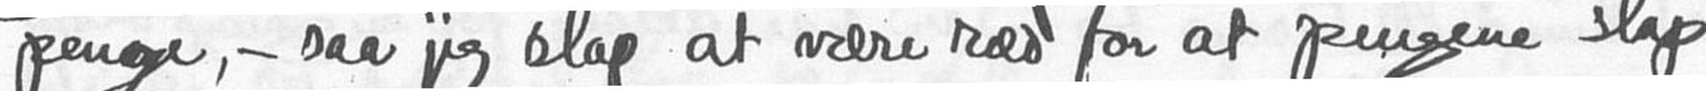penge, - saa jeg slap at være ræd for at pengene slap
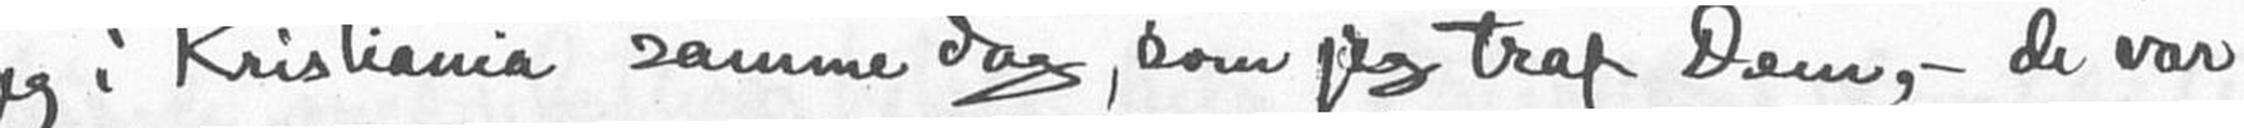jeg i Kristiania samme dag, som jeg traf Dem, - de var
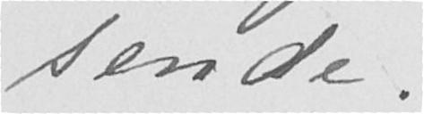sende.
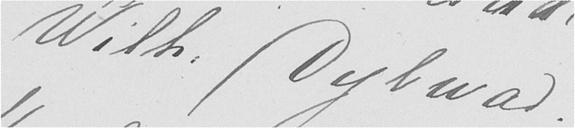Wilh. Dybwad.
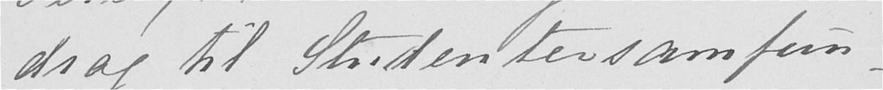drag til Studentersamfun-
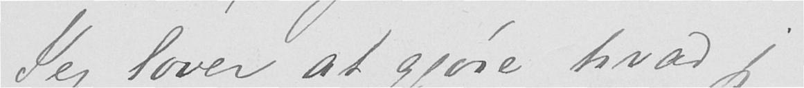Jeg lover at gjøre hvad jeg
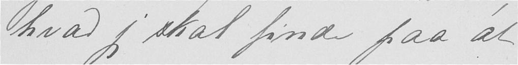hvad jeg skal finde paa at
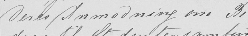Deres Anmodning om Bi-
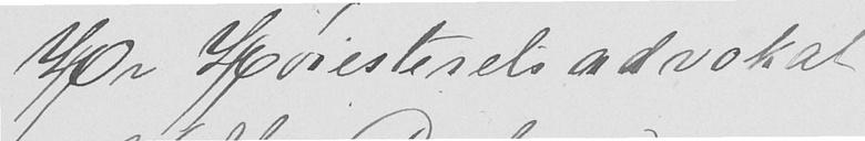Hr Høiesteretsadvokat
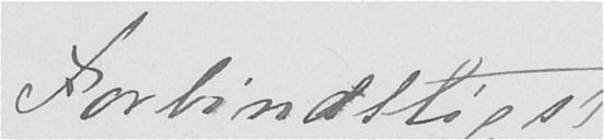Forbindtligst
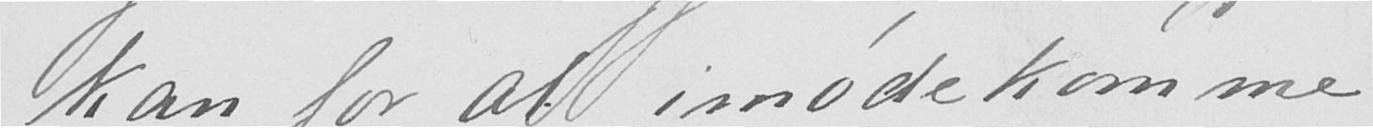kan for at imødekomme
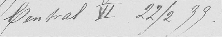Central VI  22/2 99.
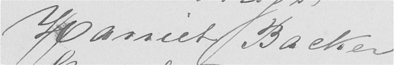Harriet Backer
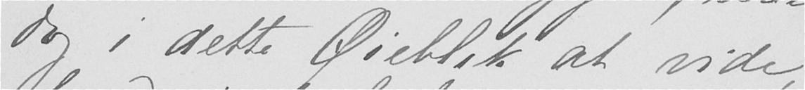dog i dette Øieblik at vide,
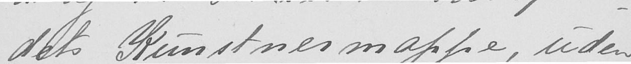dets Kunstnermappe, uden
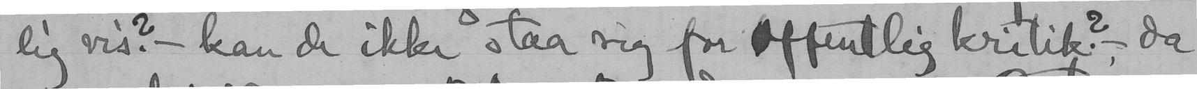lig vis? - kan de ikke staa sig for offentlig kritik?, - da
